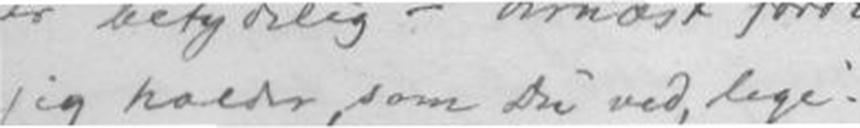jeg holder som Du ved, lige-
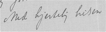Med hjertelig hilsen
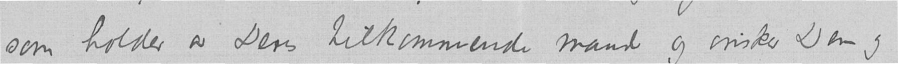som holder av Deres tilkommende mand og ønsker Dem og
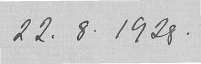22.8.1928
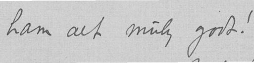ham alt mulig godt!
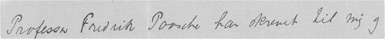Professor Fredrik Paasche har skrevet til mig og
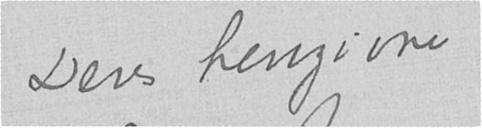Deres hengivne
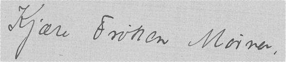Kjære Frøken Mørner,
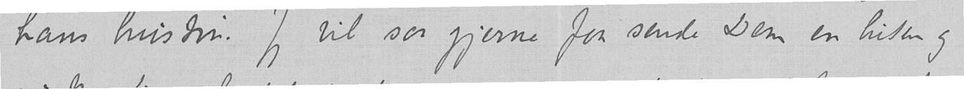hans hustru. Jeg vil saa gjerne faa sende Dem en hilsen og
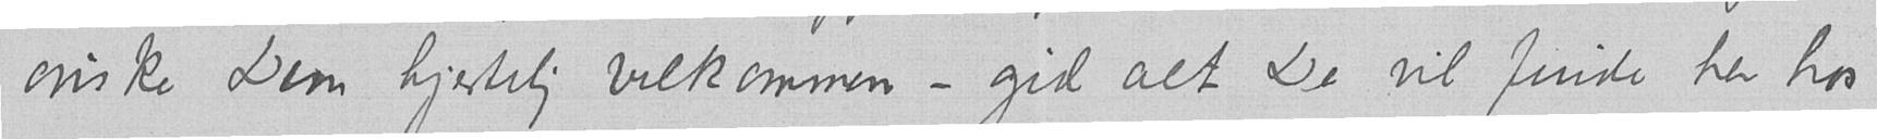ønske Dem hjertelig velkommen – gid alt De vil finde her hos
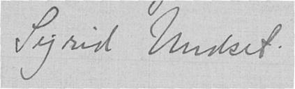Sigrid Undset.
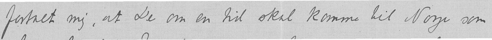fortalt mig, at De om en tid skal komme til Norge som
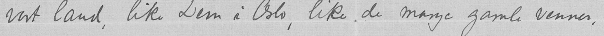vort land, like Dem i Oslo, like de mange gamle venner,
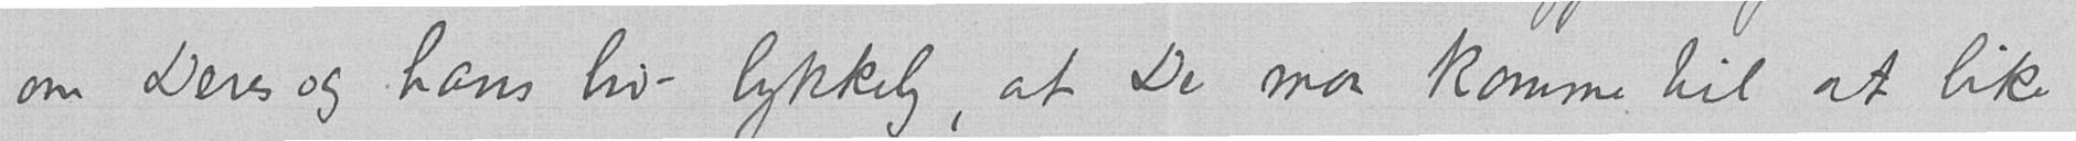om Deres og hans liv lykkelig, at De maa komme til at like
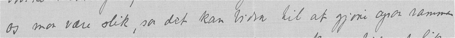os maa være slik, saa det kan bidra til at gjøre ogsaa rammen
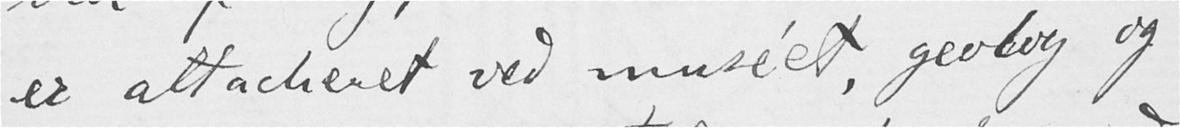er attacheret ved muséet, geolog og
